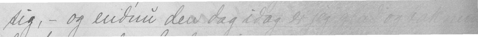sig, - og endnu den dag idag er jeg glad og teknem-
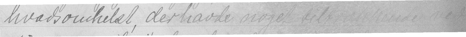hvadsomhelst, der havde noget tiltrekkende ved
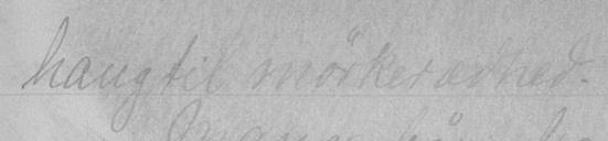hang til mørkerædhed.
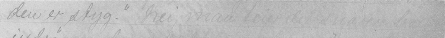den er styg." Nei, man feier det snar bort
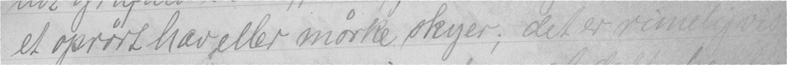et oprørt hav eller mørke skyer; det er rimeligvis
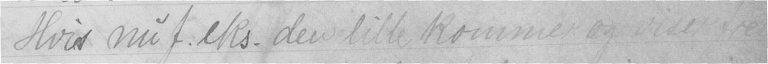Hvis nu f. eks. den lille kommer og viser frem
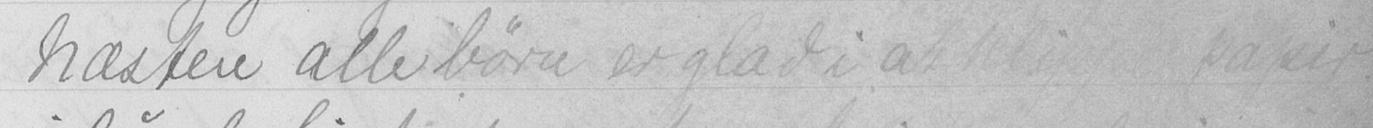Næsten alle børn er glad i at klippe papir.
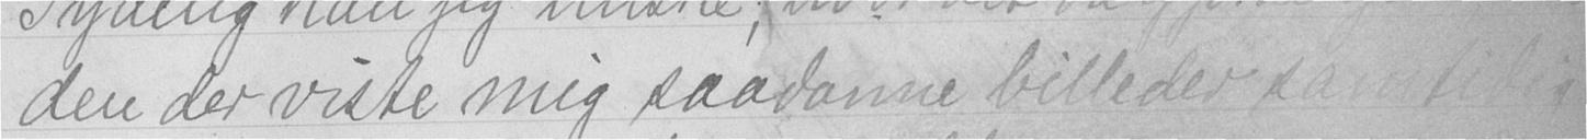den der viste mig saadanne billeder samtidig
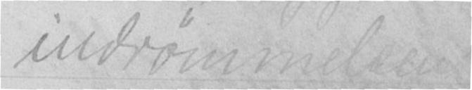indrømmelsen: "
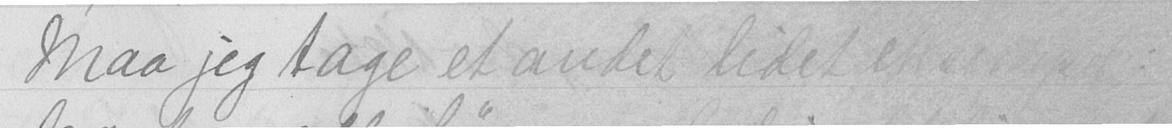maa jeg tage et andet lidet eksempel:
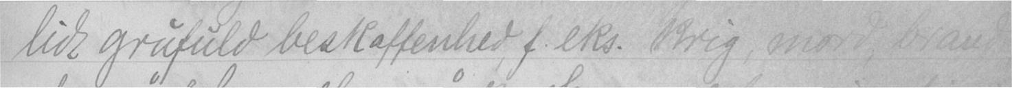lidt grufuld beskaffenhed, f. eks. krig, mord, brand,
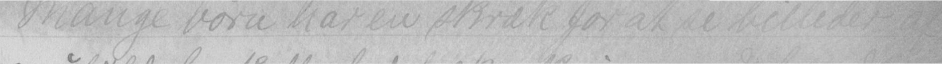Mange børn har en skræk for at se billeder af
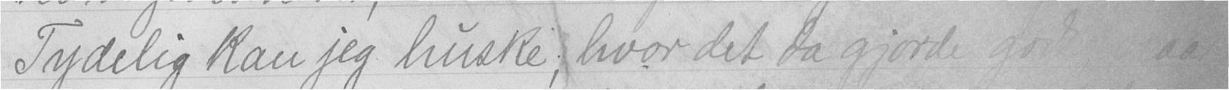Tydelig kan jeg huske, hvor det da gjorde godt naar
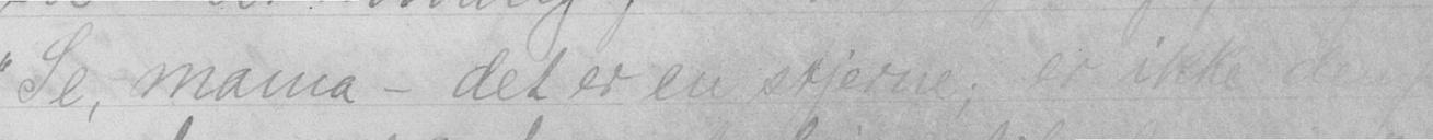"Se, mama - det er en stjerne; er ikke den
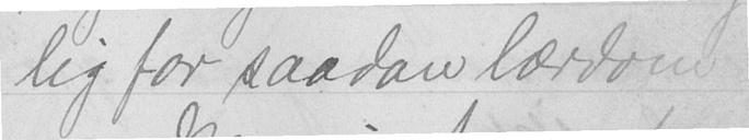lig for saadan lærdom!
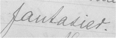fantasier.
